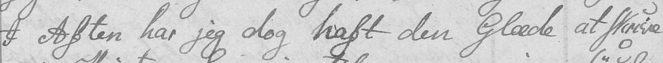I Aften har jeg dog haft den Glæde at skrive
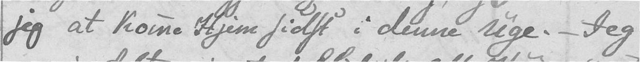jeg at komme Hjem sidst i denne Uge. – Jeg
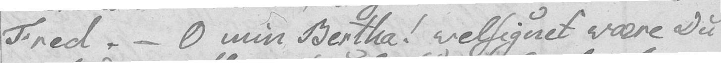Fred. – O min Bertha! velsignet være Du
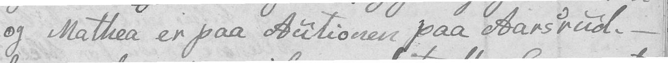og Mathea er paa Autionen paa Aarsrud. –
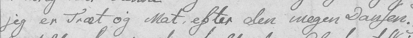jeg er Træt og Mat, efter den megen Dansen.
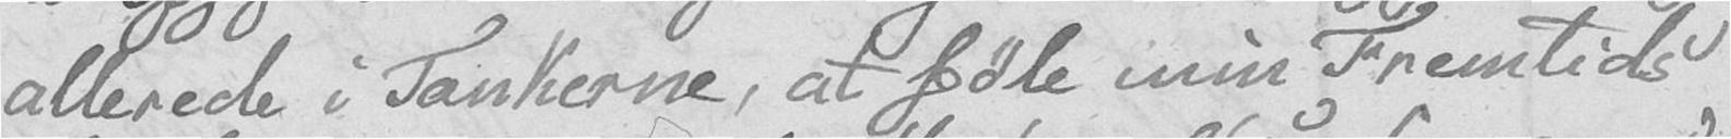allerede i Tankerne, at føle min Fremtids
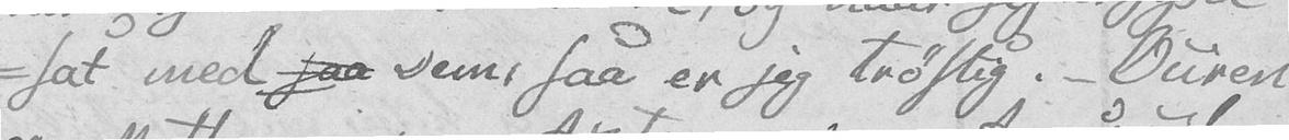-sat med saa Dem, saa er jeg trøstig. – Ouren
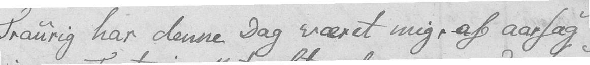Traurig har denne Dag været mig, af aarsag
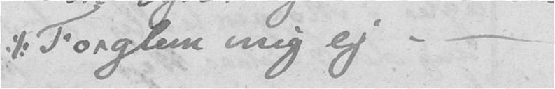:/: Forglem mig ej. –
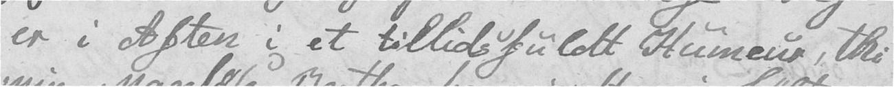er i Aften i et tillidsfuldt Humeur, thi
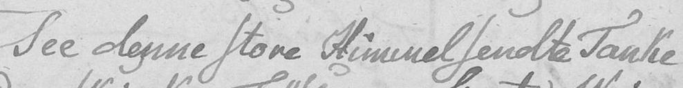See denne store Himmelsendte Tanke
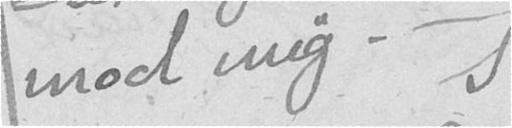mod mig. –
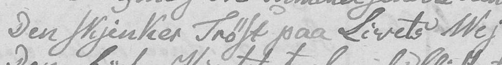Den skjenker Trøst paa Livets Vei
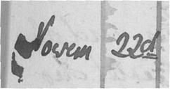Novem 22d
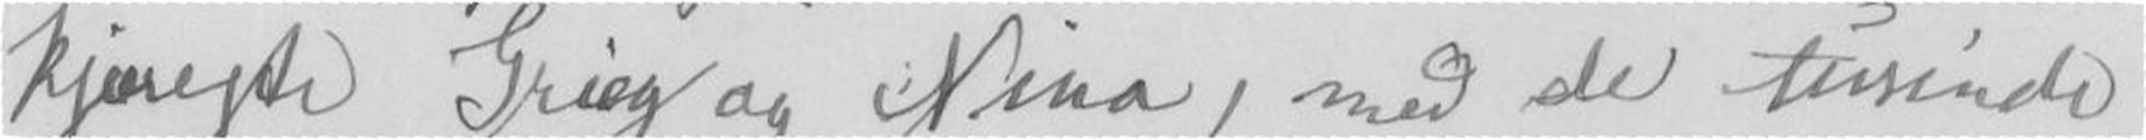kjæreste Grieg og Nina, med de tusinde
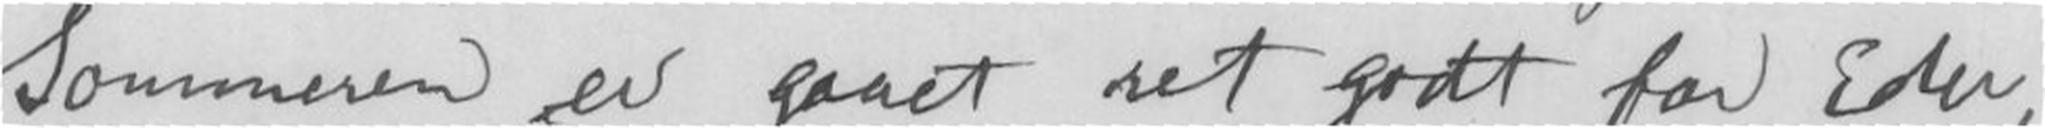Sommeren er gaaet ret godt for Eder,
